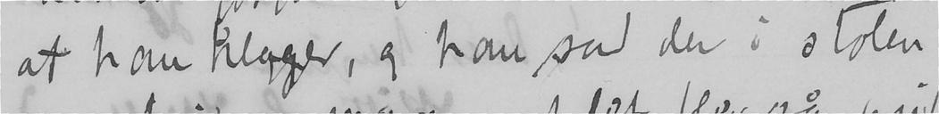at han klager, og han sad der i stolen
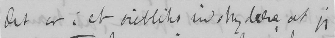Det er i et øiebliks indskydelse, at jeg
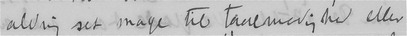aldrig set mage til taalmodighed eller
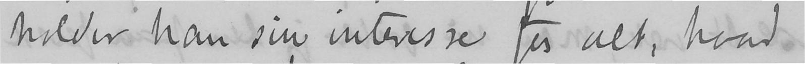holder han sin interesse for alt, hvad
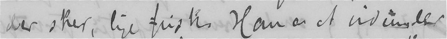der sker, lige frisk. Han er et vidunder
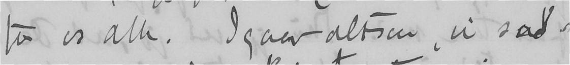for os alle. Igaar altsaa, vi sad'
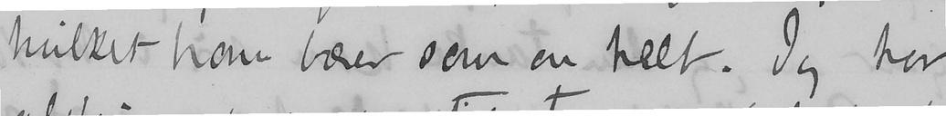hvilket han bærer som en helt. Jeg har
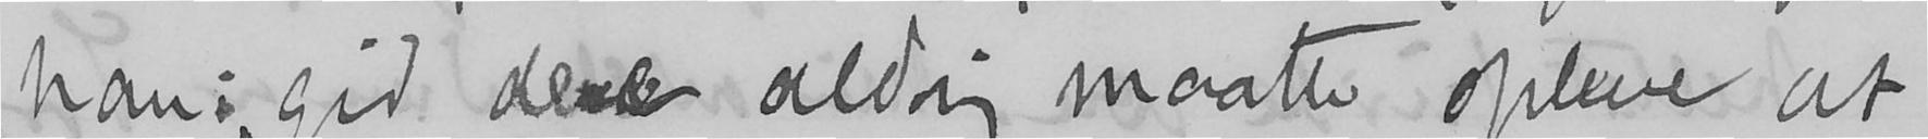han: "gid dere aldrig maatte opleve, at
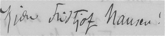Kjære Fridtjof Nansen!
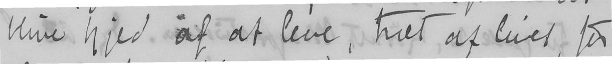blive kjed af at leve, træt af livet, for
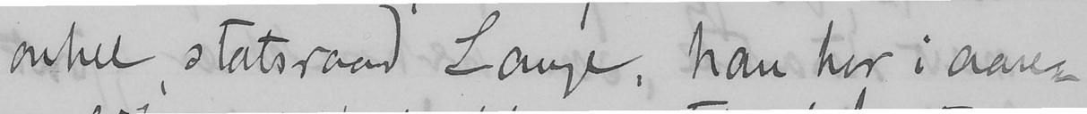onkel, statsraad Lange, han har i aare-
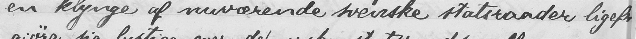en klynge af nuværende svenske statsraader ligefrem
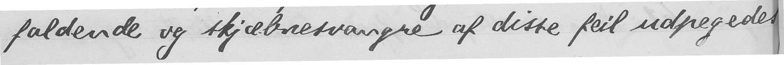faldende og skjæbnesvangre af disse feil udpegedes
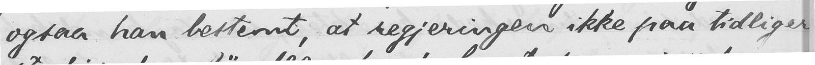ogsaa han bestemt, at regjeringen ikke paa tidligere
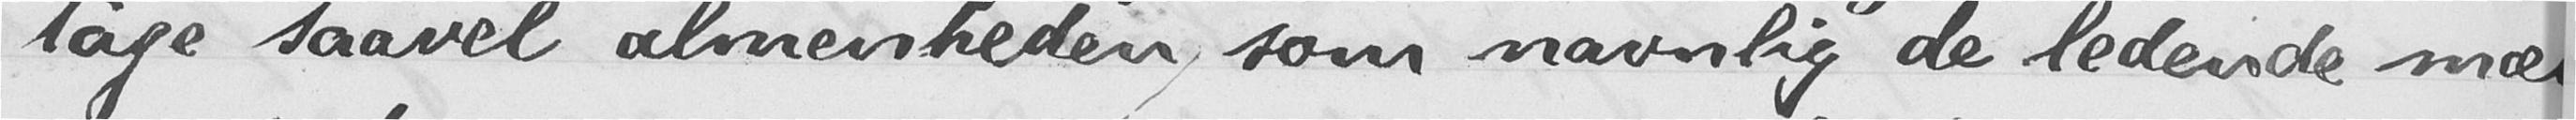tage saavel almenheden som navnlig de ledende mænd
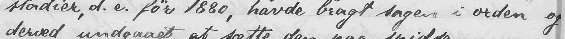stadier, d. e. før 1880, havde bragt sagen i orden og
